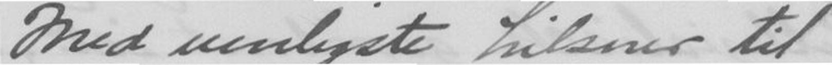Med venligste hilsner til
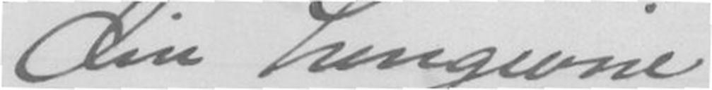Din hengivne
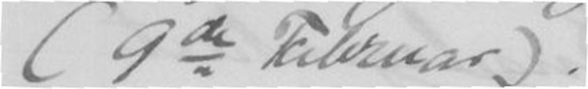(9de Februar).
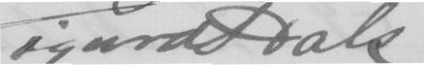Sigurd Hals.
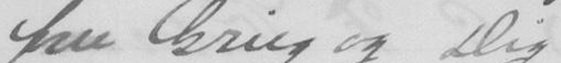fru Grieg og Dig
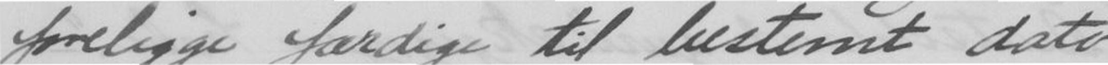foreligge færdige til bestemt dato
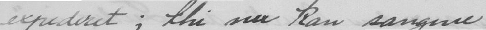expederet; thi nu kan sangene
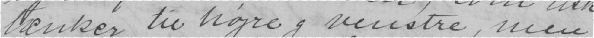tænker til höjre og venstre, men
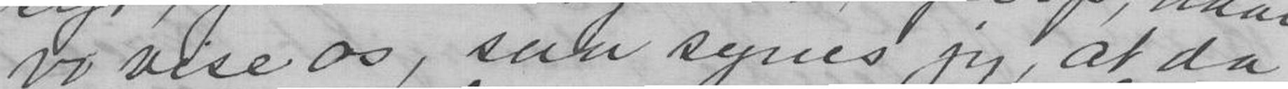vi vise os, saa synes jeg, at da
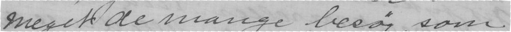meget de mange besög, som
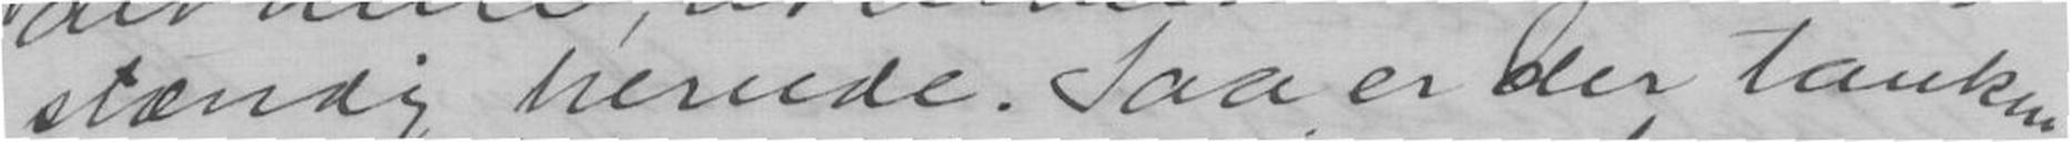stændig hernede. Saa er der tanken
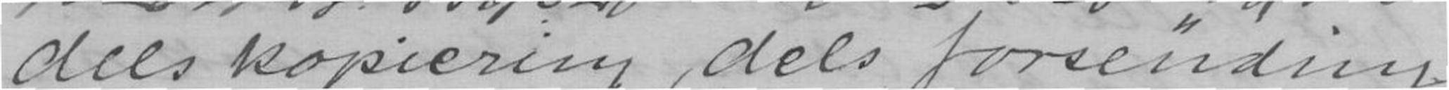dels kopiering, dels forsending,
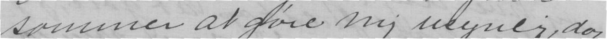sommer at göre mig usynling, dog
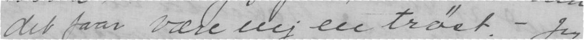det faar være mig en tröst.- Jeg
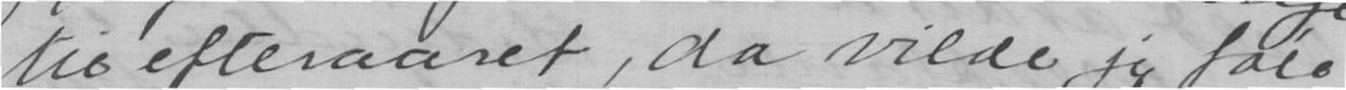til efteraaret, da vilde jeg føle
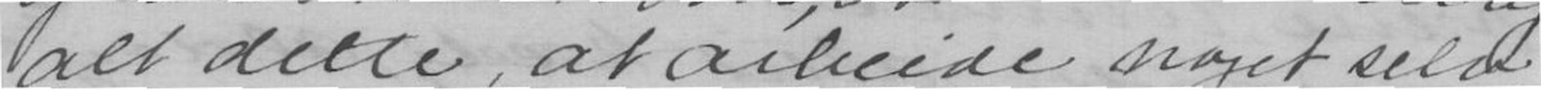alt dette, at arbeide noget selv-
